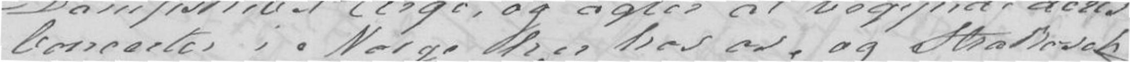Concerter i Norge her hos os, og Strakosch
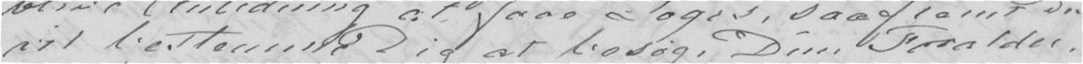vil bestemme Dig at besøge Dine Forældre,
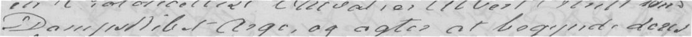Dampskibet Argo, og agter at begynne deres
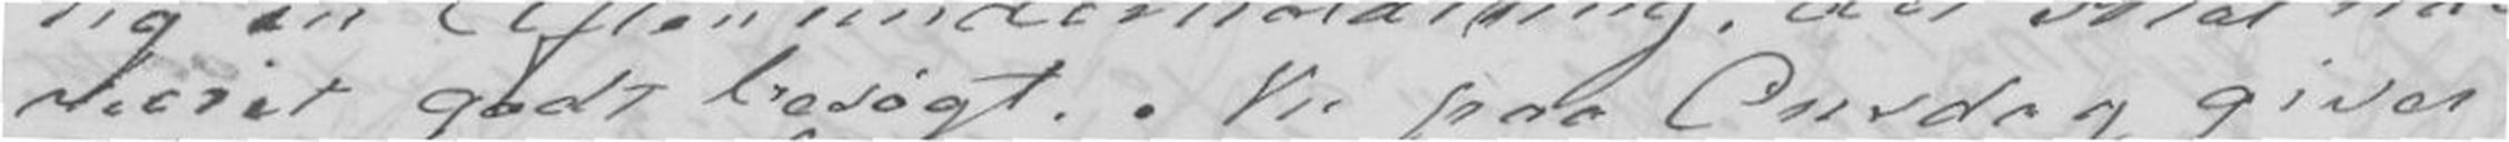værit godt besøgt. Nu paa Onsdag giver
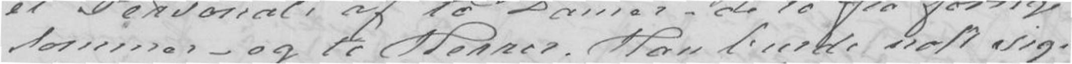sommer - og to Herrer. Hun burde nok sige
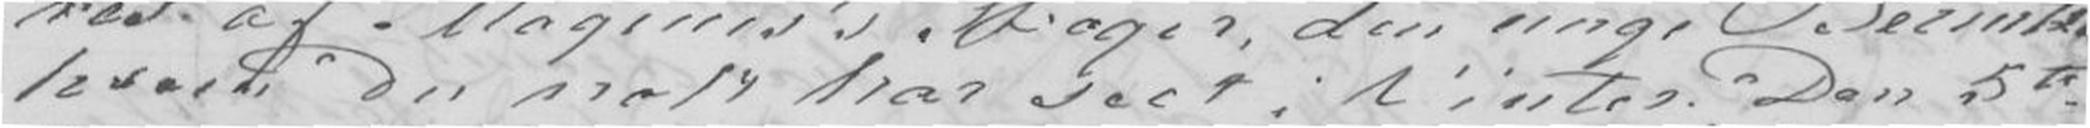hvem Du nok har seet i Vinter. Den 5te
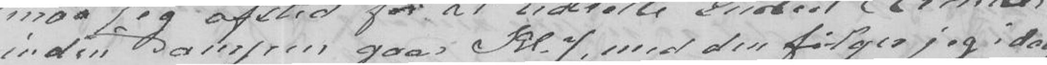inden Dampen gaar Kl.7, med den følger jeg i dag
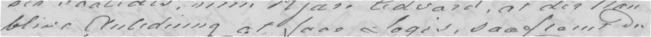blive Anledning at faae Logis saafremt Du
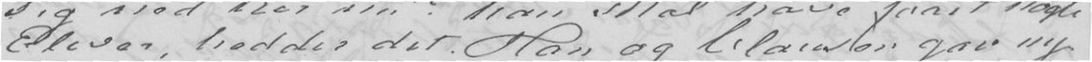Elever, hedder det. Han og Clausen gav ny-
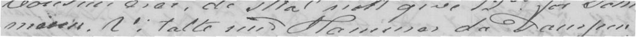meren. Vi talte med Hammer da Dampen
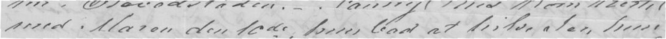med Maren den 10de, hun bad at hilse Jer, hun
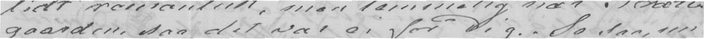gaarden, som det var ei for Dig. Se saa, nu
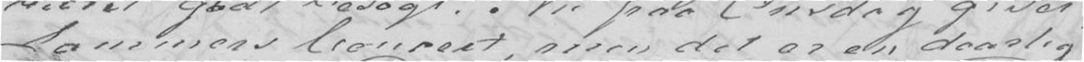Lammers Concert, men det er en daarlig
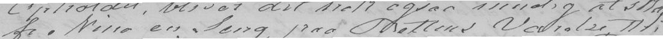fe Nina en Seng paa Bettens Værelse, thi
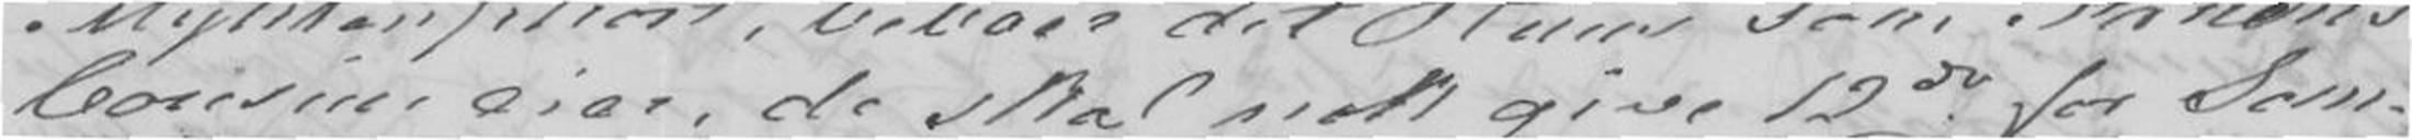Cousine eier, de skal nok give 12do for Som-
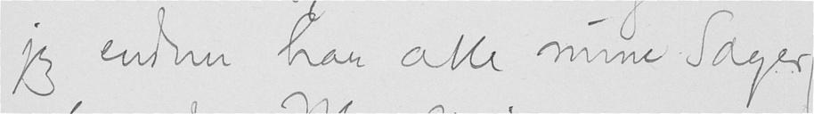jeg endnu har alle mine Sager
